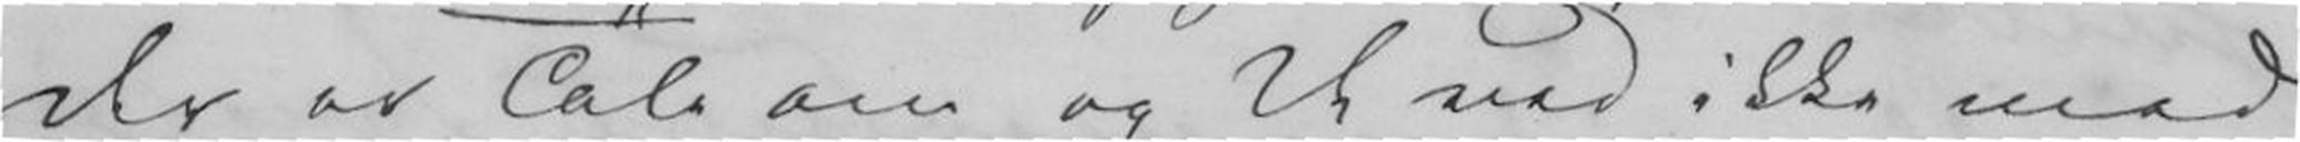der er Tale om og De ved ikke med
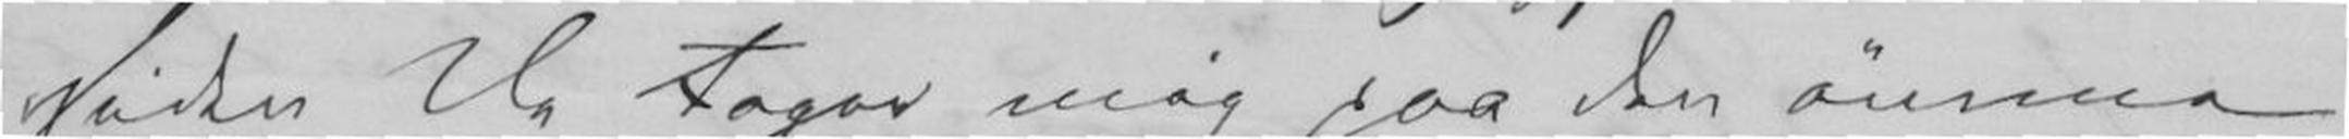siden De tager mig paa den ømme
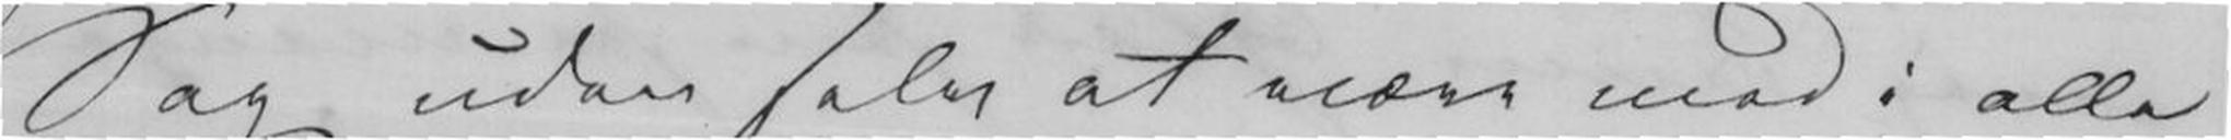Sag uden selv at være med i alle
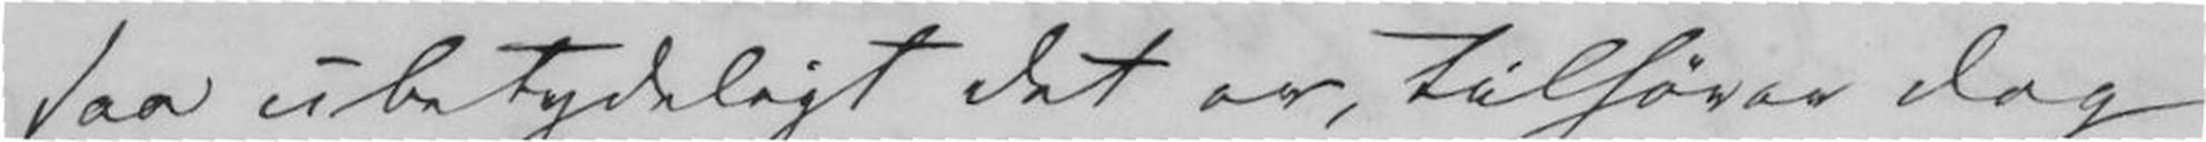saa ubetydeligt det er, tilhører dog
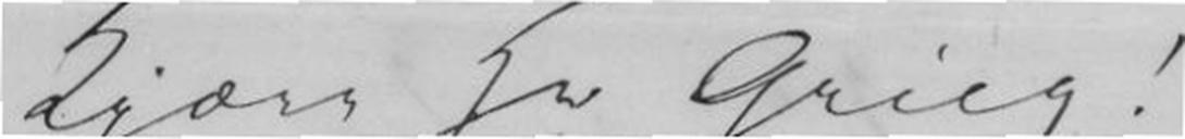Kjære Hr. Grieg!
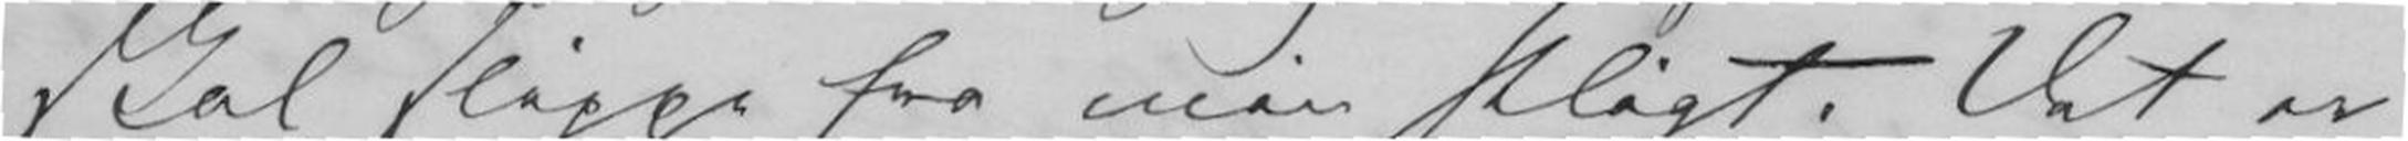skal slippe fra min pligt. Det er
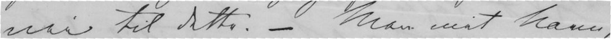nei til ditto. - Men mit Navn,
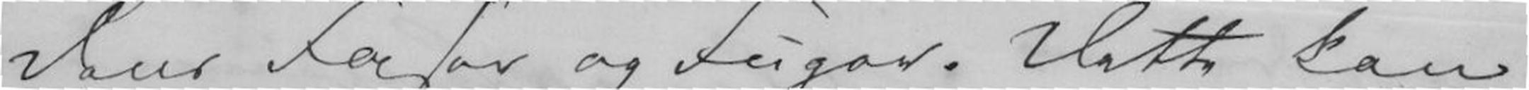dens Faser og Fuger. Dette kan
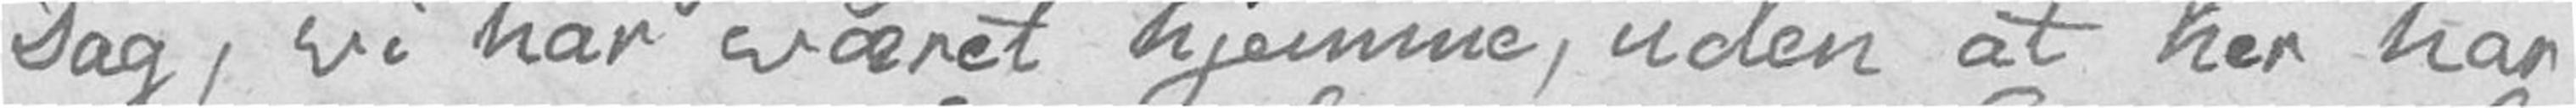Dag, vi har været hjemme, uden at her har
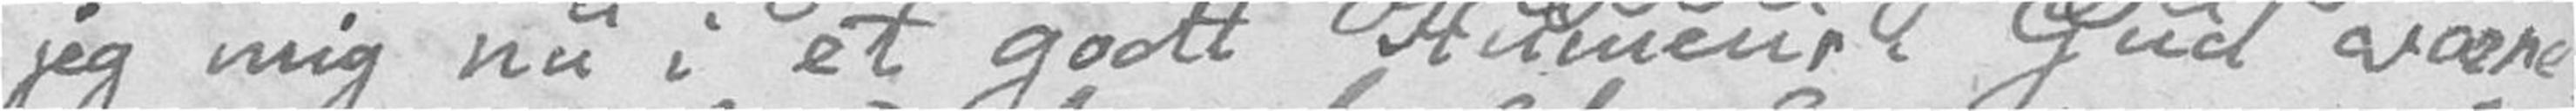jeg mig nu i et godt Humeur. Gud være
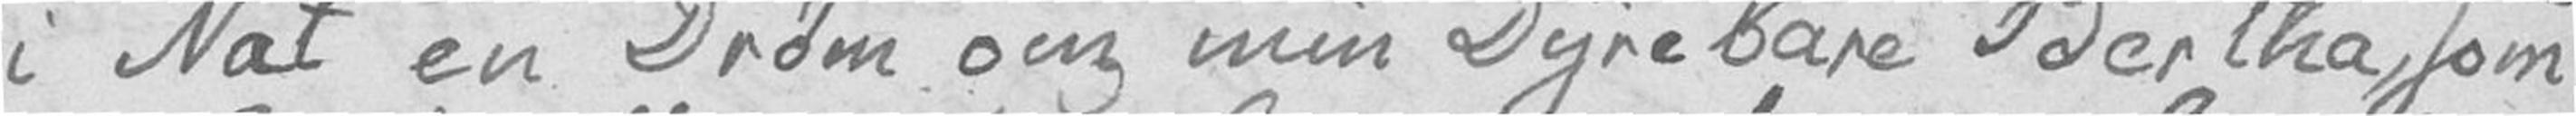i Nat en Drøm om min Dyrebare Bertha, som
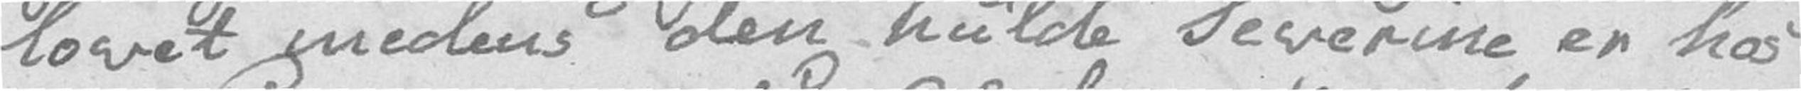lovet medens den hulde Severine er hos
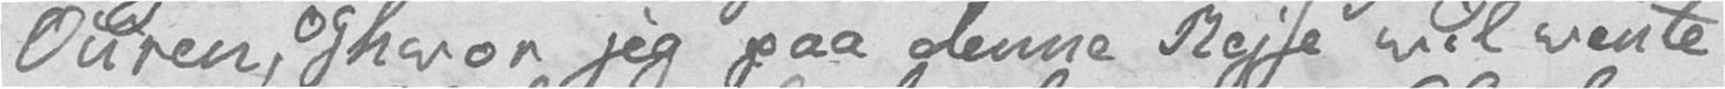Ouren, og hvor jeg paa denne Rejse vil vente
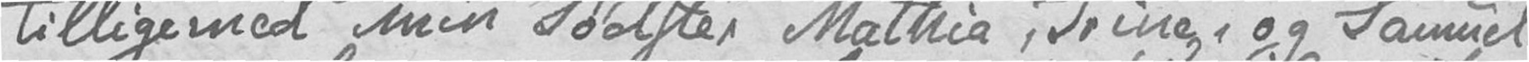tilligemed min Sødster Mathia, Trine, og Samuel
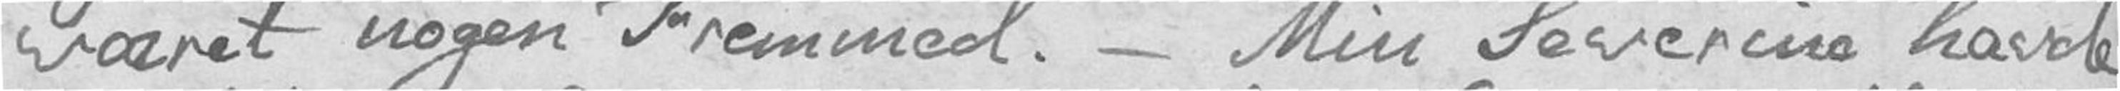været nogen Fremmed. – Min Severine havde
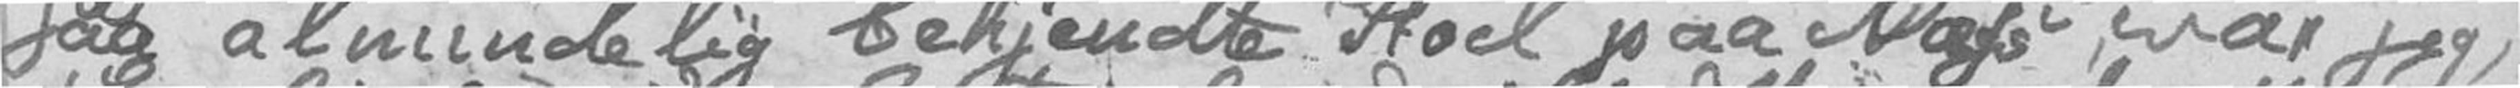saa almindelig bekjendte Hoel paa Næss, var jeg,
